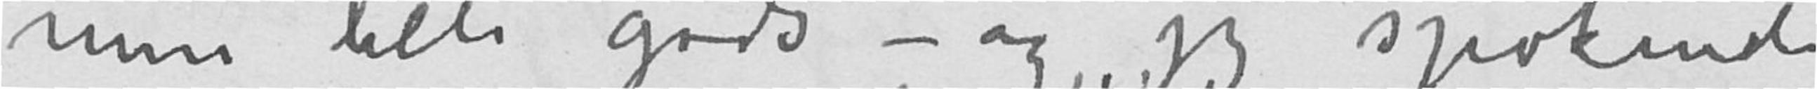min lille gods – og jeg spøkende
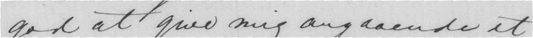god at give mig angaaende et
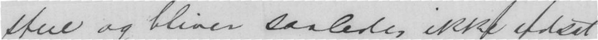stue og bliver saaledes ikke udsa t
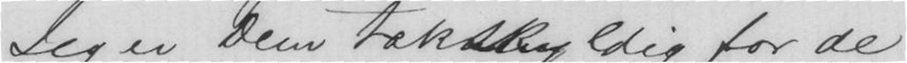Jeg er Dem takskyldig for de
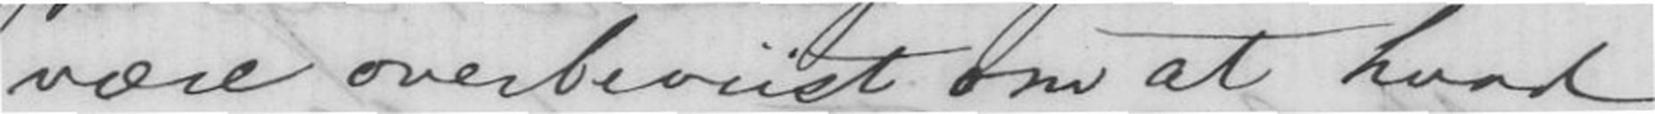være overbevist om at hvad
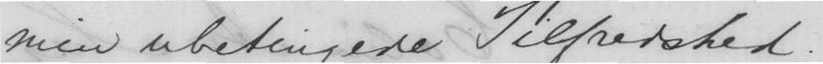min ubetingede Tilfredshed.
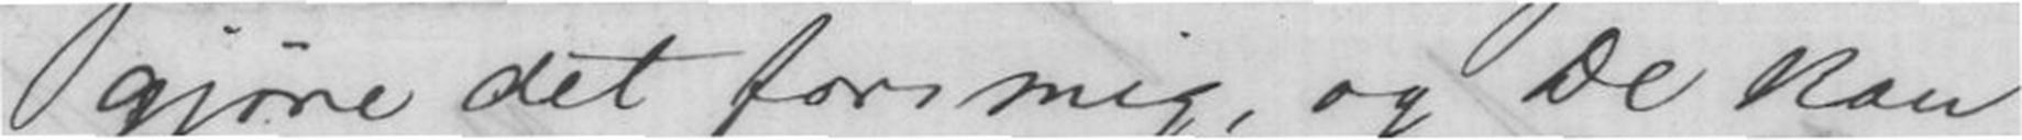gjøre det for mig, og De kan
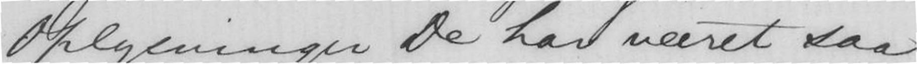Oplysninger De har været saa
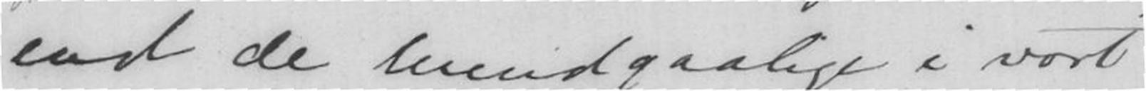end de Uungaalige i vort
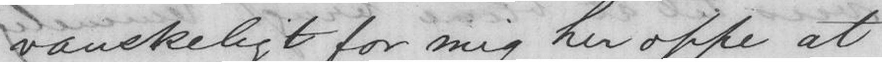vanskeligt for mig her oppe at
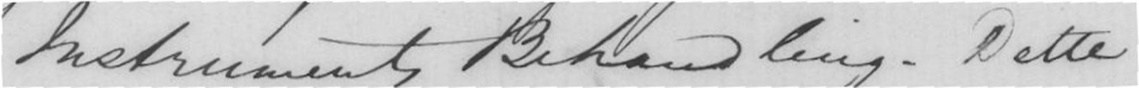Instrument Behandling. Dette
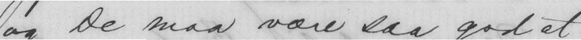og De maa være saa god at
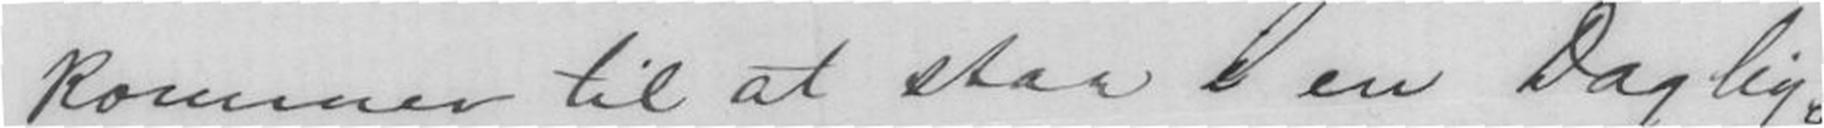kommer til at staa i en Daglig-
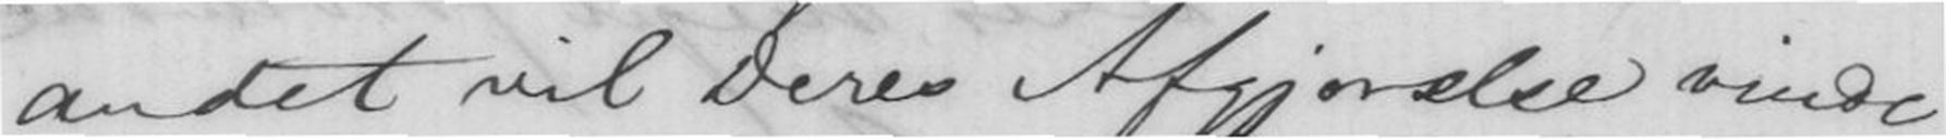andet vil Deres Afgjørelse vinde
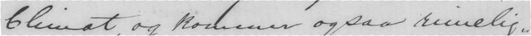Climat, og kommer ogsaa rimelig-
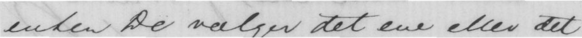enten De vælger det ene eller det
